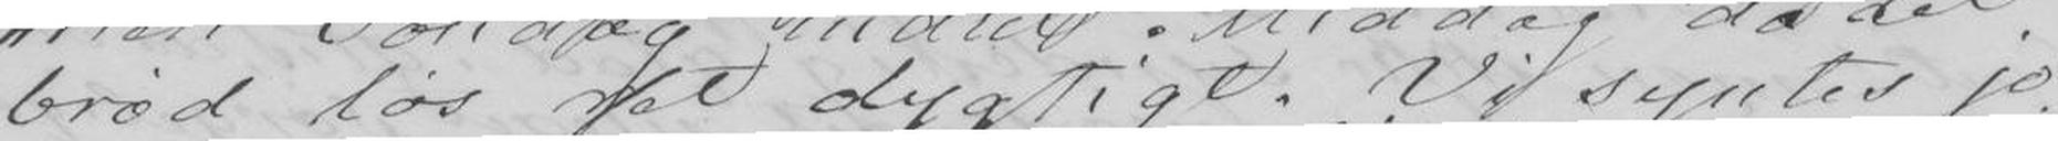brød løs ret dygtig. Vi syntes jo
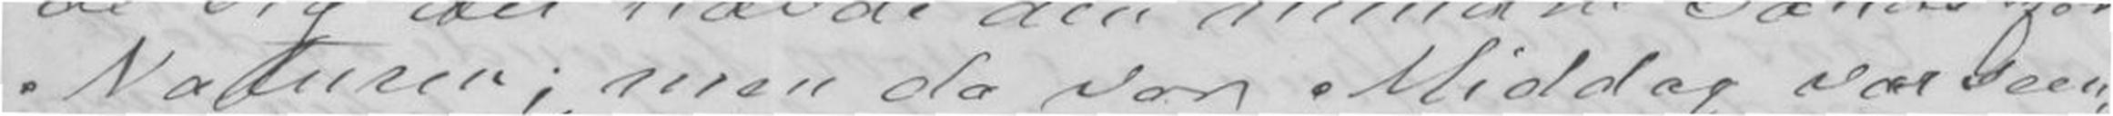Naturen; men da vor Middag var seen,
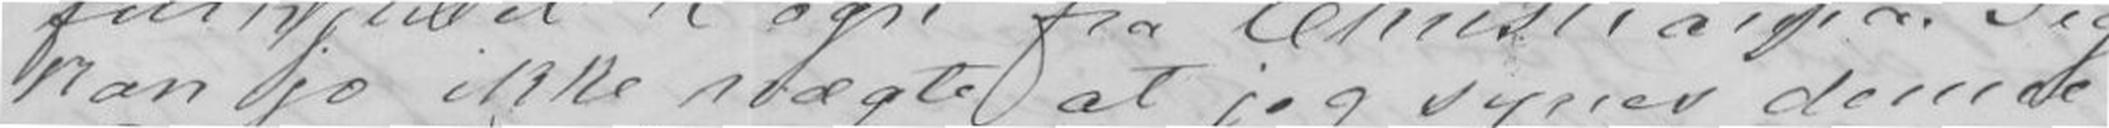kan jo ikke nægte at jeg synes denne
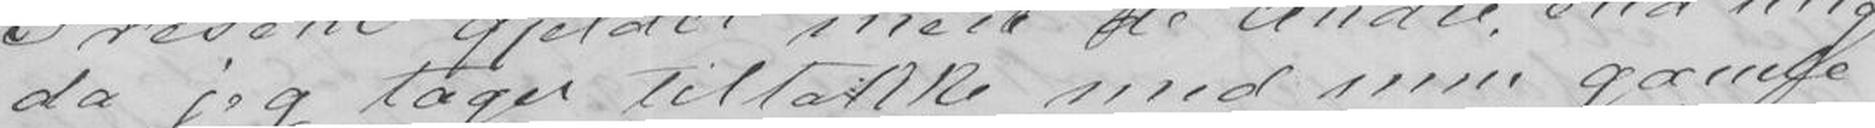da jeg tager tiltakke med min gamle
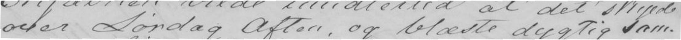over Lørdag Aften, og blæste dygtig sam-
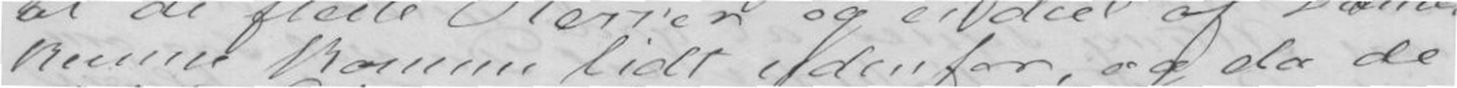kunne komme lidt udenfor, og da de
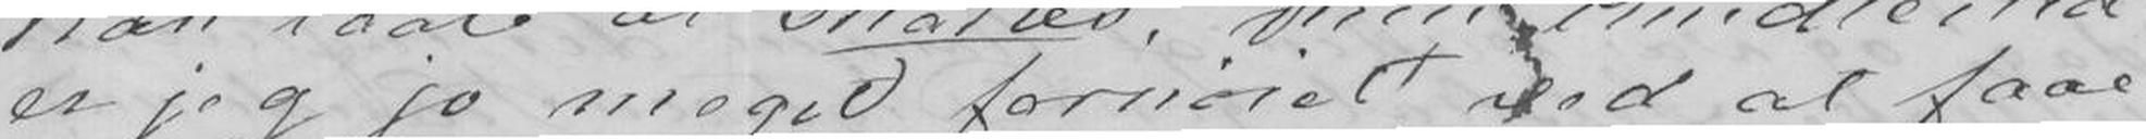er jeg jo meget fornøiet ved at faae
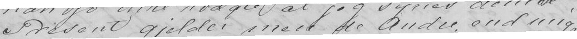Present gjelder mere de Andre end mig,
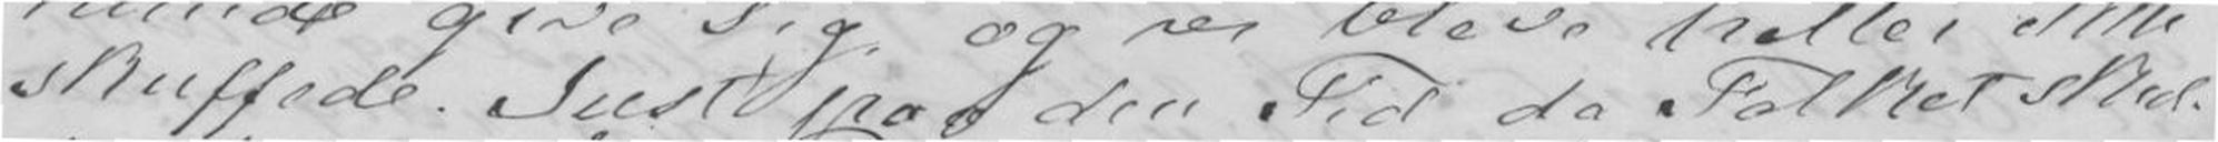skuffede. Just paa den Tid da Folket skul-
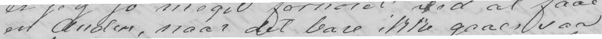en Anden, naar det bare ikke gaaer saa
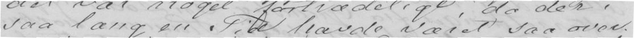saa lang en Tid havde været saa over-
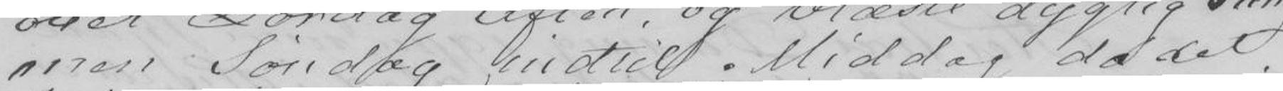men Søndag indtil Middag da det
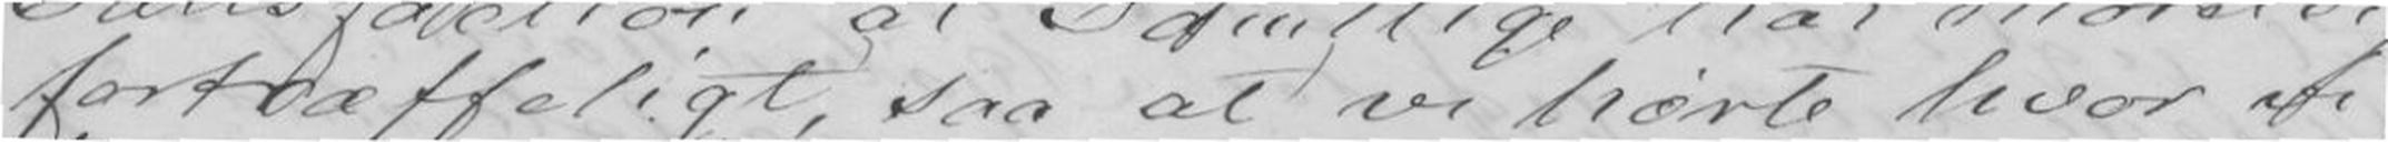fortræffeligt, saa at vi hørte hvor vi
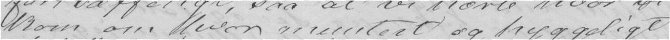kom om hvor muntert og hyggeligt
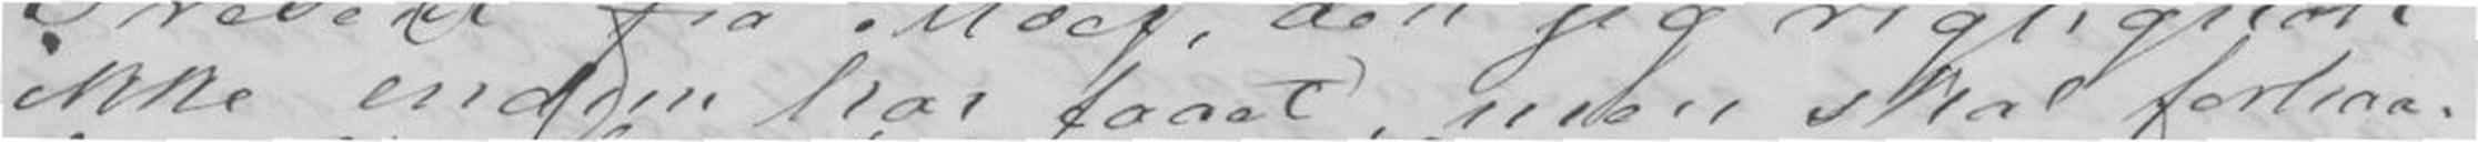ikke endnu har faaet, men skal forhaa-
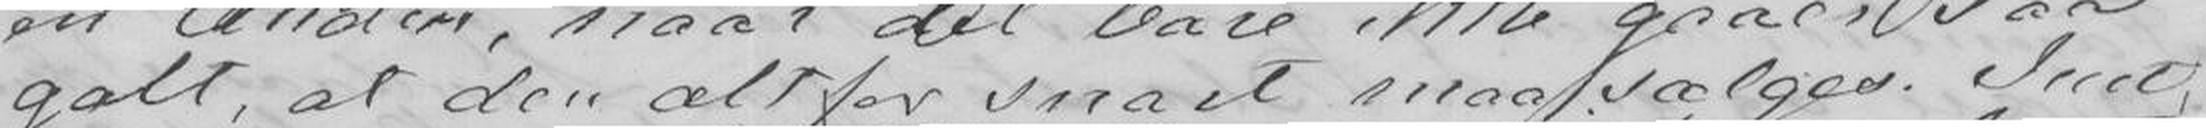galt, at den altfor snart maa sælges. Just
